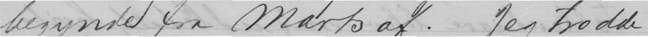begynde fra Marts af. Jeg trodde
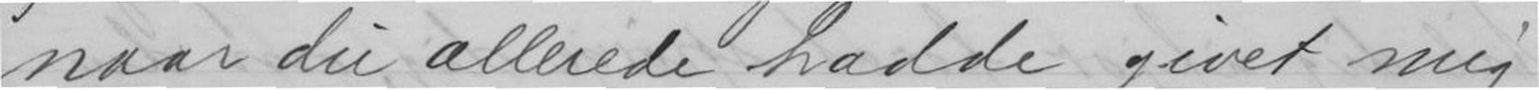naar du allerede hadde givet mig
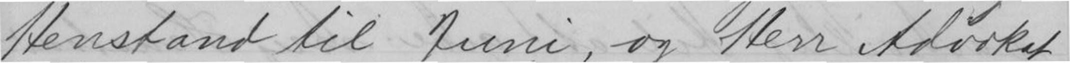Henstand til Juni, og Herr Advokat
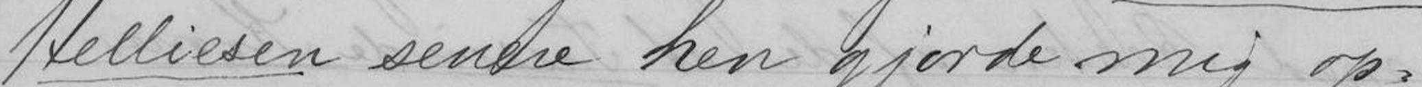Helliesen senere her gjorde mig op-
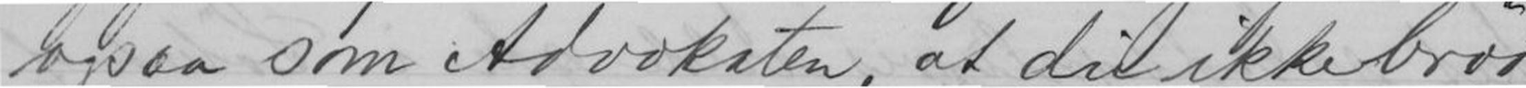ogsaa som Advokaten, at du ikke brød
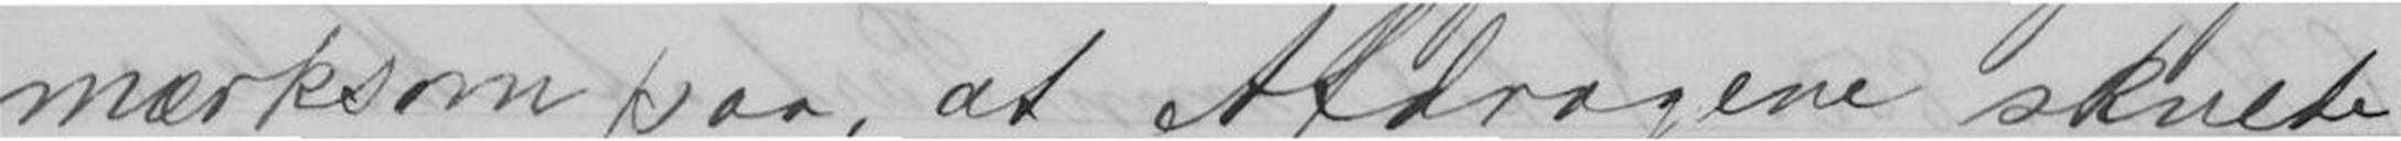mærksom paa, at Afdragene skulde
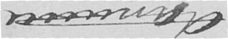danuevel
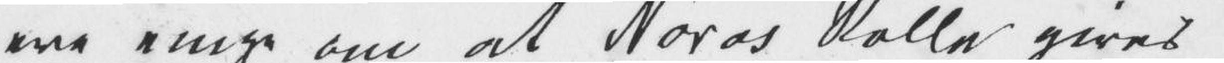ere enige om at Noras Rolle gives
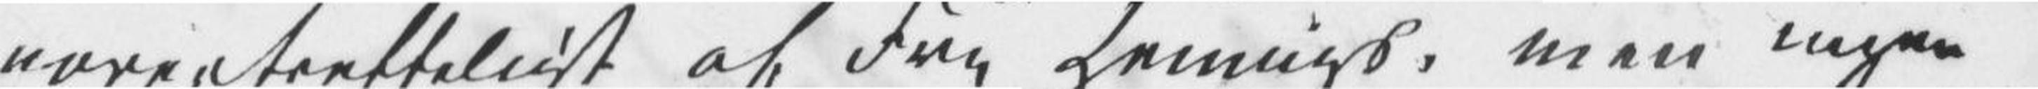uovertreffeligt af Fru Hennings, men ingen
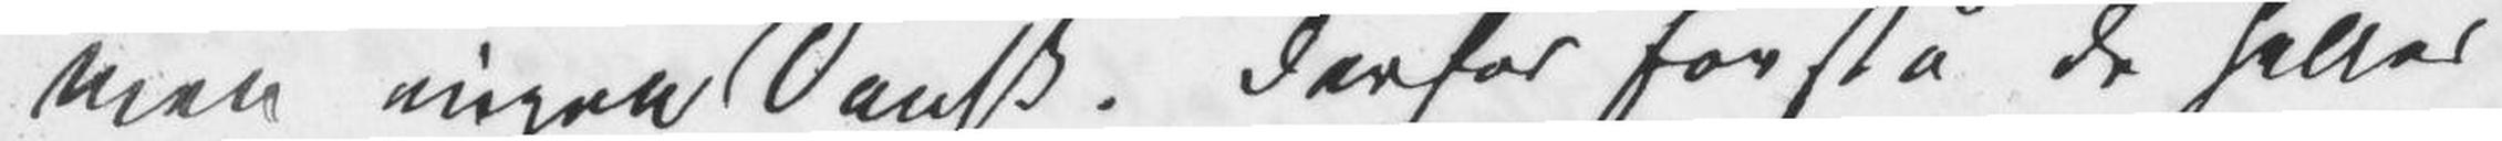men ingen Dansk. Derfor forstå de heller
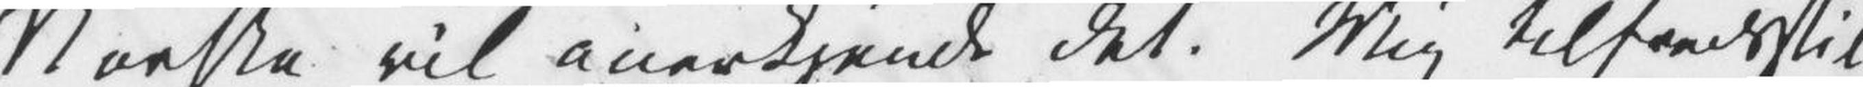Norske vil anerkjende det. Mig tilfredsstil¬
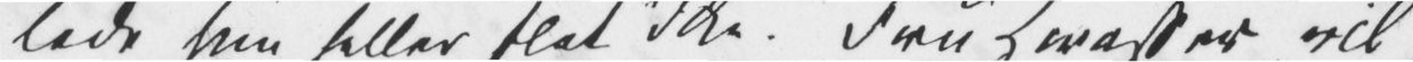lede hun heller slet ikke. Fru Hwasser vil
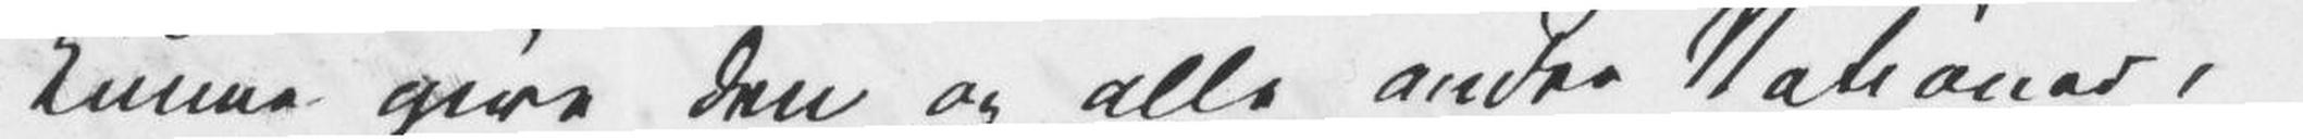kunne give den og alle andre Nationer,
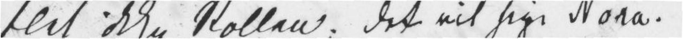slet ikke Rollen; det vil sige Nora.
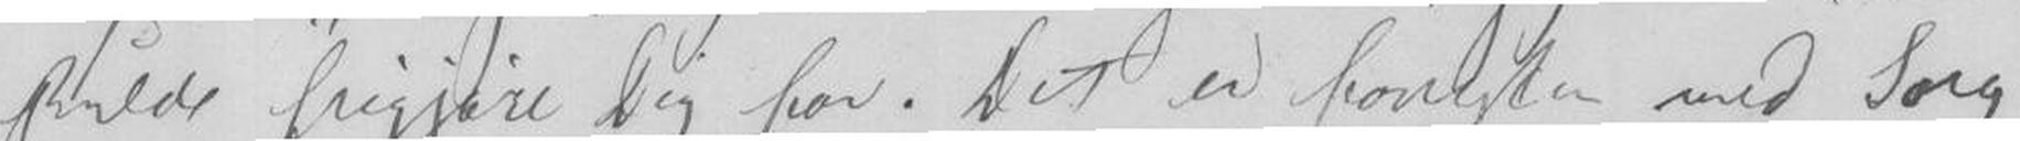skulde frigjøre Dig for. Det er forresten med Sorg
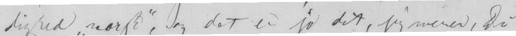dighed norsk, og det er jo det, jeg mener, Du
